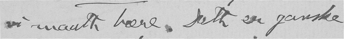vi maatte bære. Dette er ganske
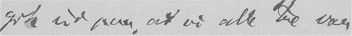gik ud paa, at vi alle tre var
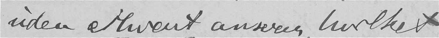uden ethvert ansvar, hvilket
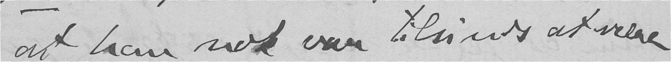at han nok var tilsinds at være
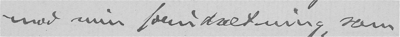mod min forudsætning, som
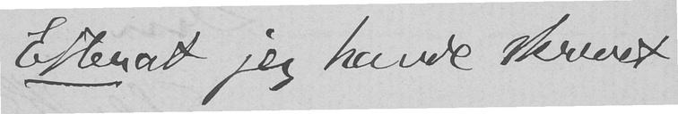Efterat jeg havde skrevet,
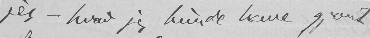jeg - hvad jeg burde have gjort
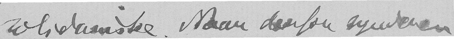solidariske. Naar derfor synderen
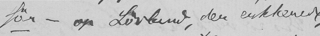før - op Løvland, der erklærede,
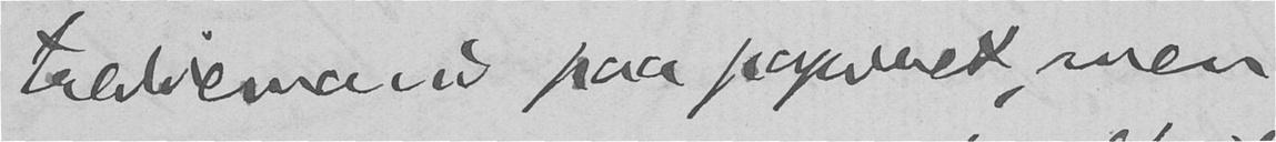trediemand paa papiret, men
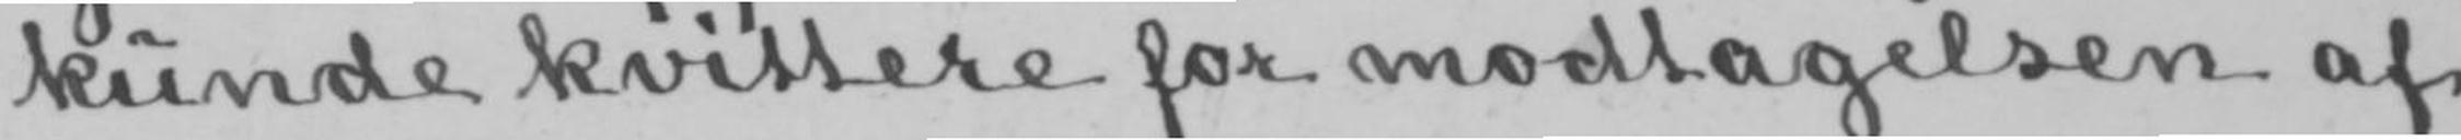kunde kvittere for modtagelsen af
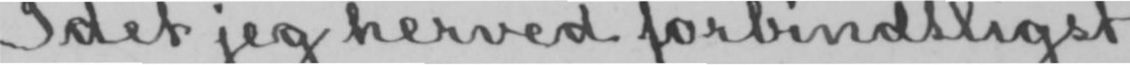Idet jeg herved forbindtligst
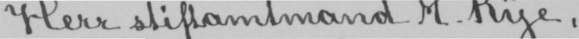Herr stiftamtmand M. Rye,
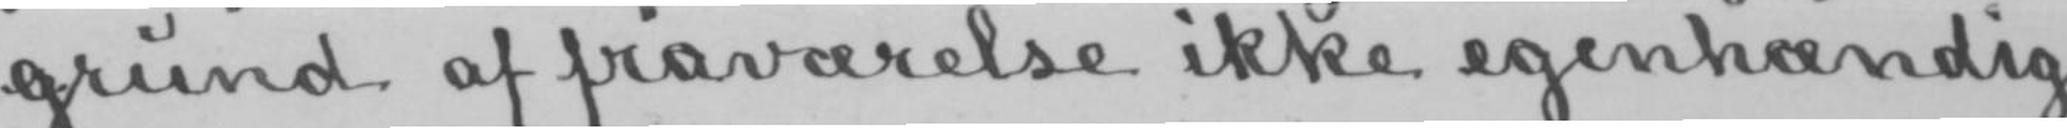grund af fraværelse ikke egenhændig
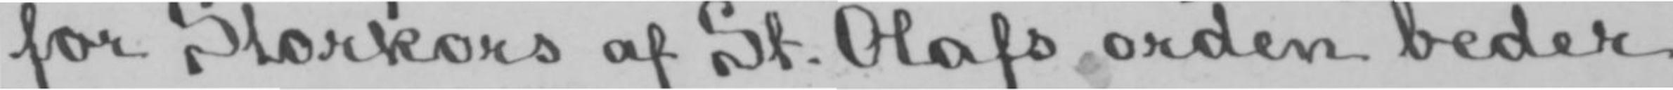for Storkors af St. Olafs orden beder
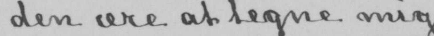den ære at tegne mig
